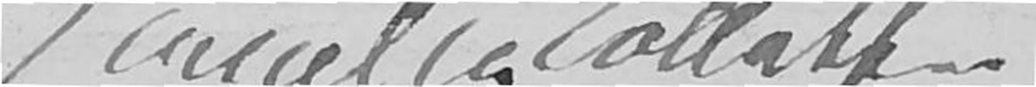Camilla Collett.
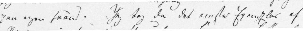paa egen Haand. Jeg tog da det eneste Exemplar af
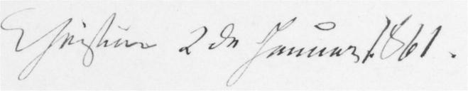Christiania 2den Januar 1861.
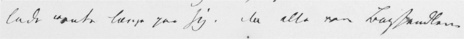lade vente længe paa sig, da alle vore Boghandlere
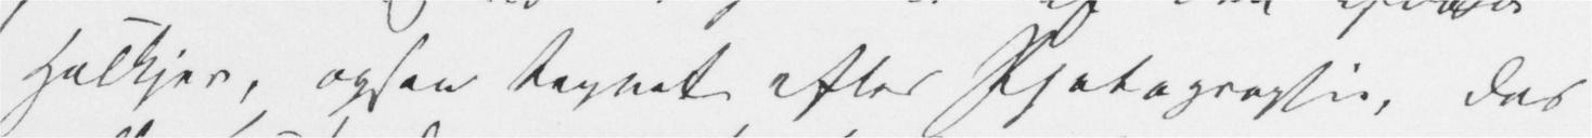Halkjer, ogsaa tegnet efter Photographie, der
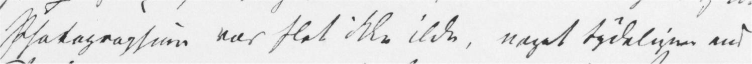Photographien var slet ikke ilde, noget tydeligere end
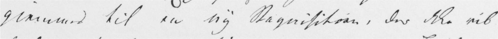gjemmes til en ny Reqvisition, der ikke vil
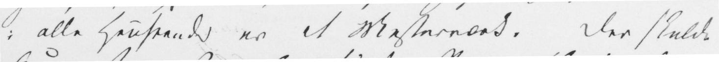i alle Henseende er et Mesterværk. Der skulde
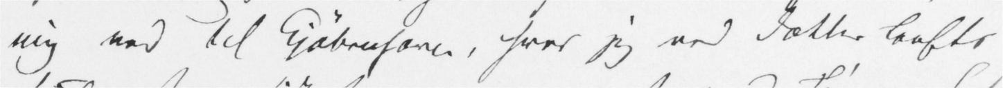mig ned til Kjøbenhavn, hvor jeg ved Fætter Loofts
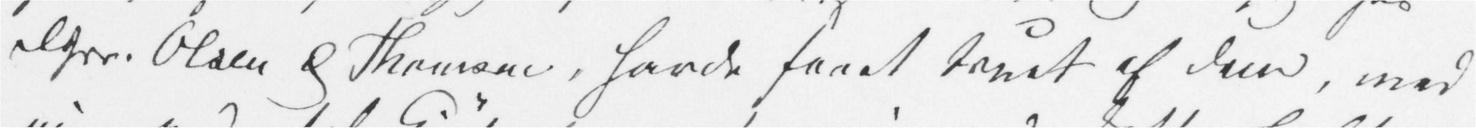Dhrr. Olsen &amp; Thomsen, havde faaet truet af dem, med
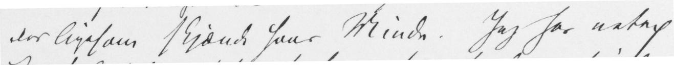der ligesom skjænde hans Minde. Jeg har netop
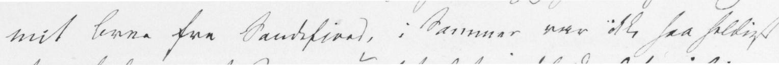mit Brev fra Sandefjord, i Sommer var ikke saa heldigt
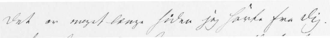Det er meget længe siden jeg hørte fra Dig.
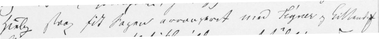Hjelp strax fik Sagen arrangeret med Tégner og Kittendorf
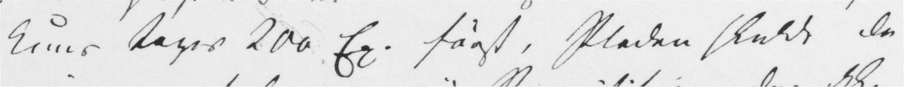kuns tages 200 Ex. først, Pladen skulde da
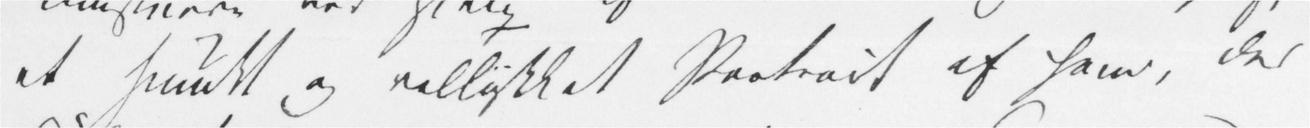et smukt og vellykket Portrait af ham, der
